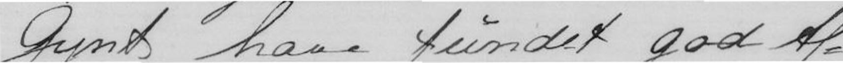Gynt have fundet god Af-
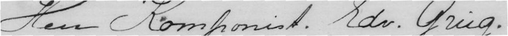Herr Komponist. Edv. Grieg.
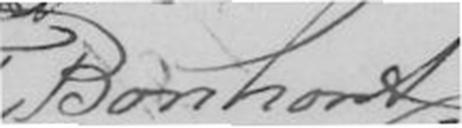F. Borchorst.
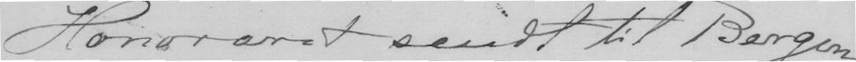Honoraret sendt til Bergen.
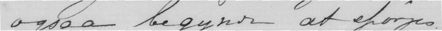ogsaa begynde at spørges.
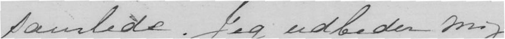samlede. Jeg udbeder mig
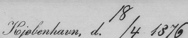Kjøbenhavn d. 18/4 1876
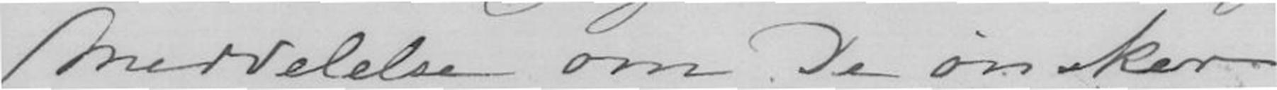Meddelse om De ønsker
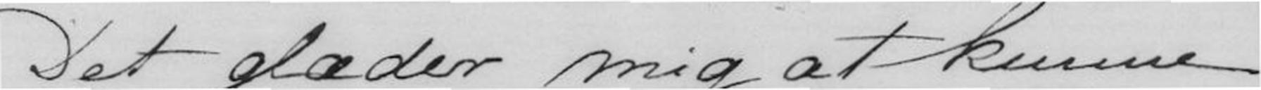Det glæder mig at kunne
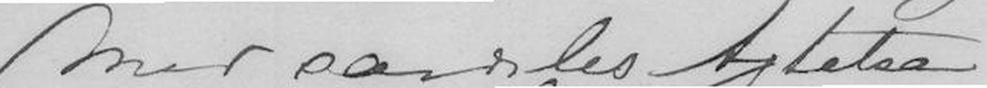Med særdeles Agtelse
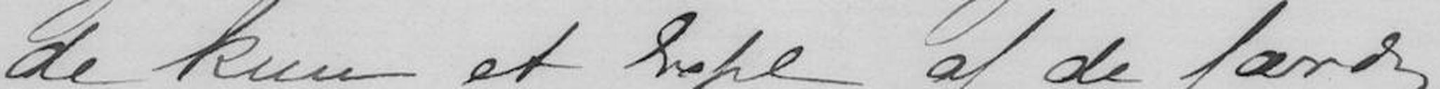de kun et Expl af de færdig
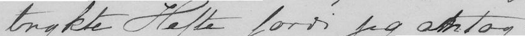trygte Hefte fordi jeg antog
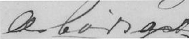Ærbødigst
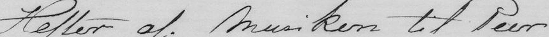Hefter af Musiken til Peer
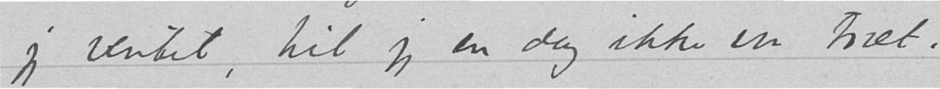jeg ventet, til jeg en dag ikke er træt.
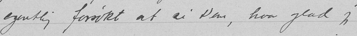egentlig forsøkt at si Dem, hvor glad jeg
